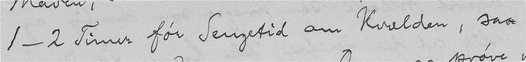1-2 Timer før Sengetid om Kvælden, saa
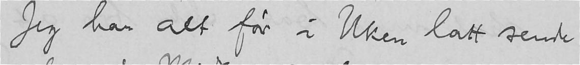Jeg har alt før i Uken latt sende
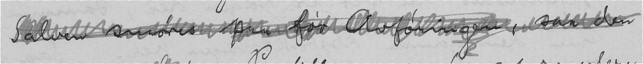Salven smøres paa før Avføringen, saa der
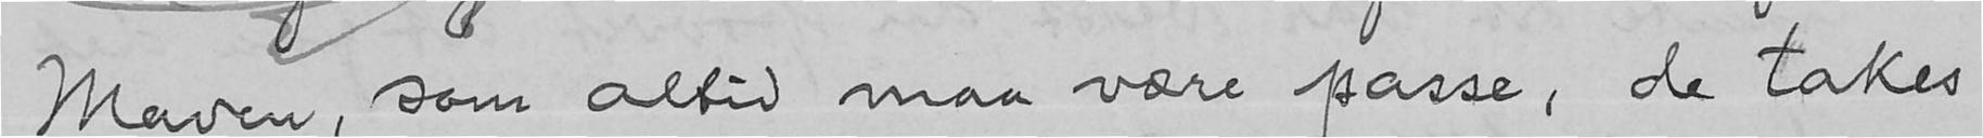Maven, som altid maa være passe, de takes
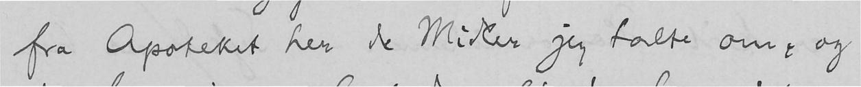fra Apoteket her de Midler jeg talte om, og
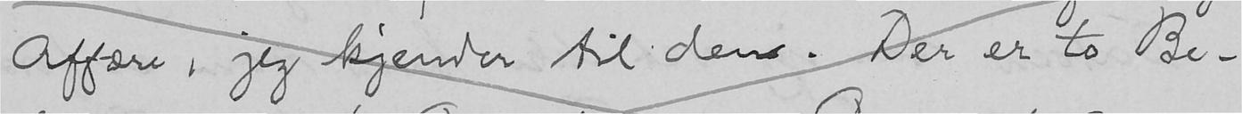Affære, jeg kjender til den. Der er to Be-
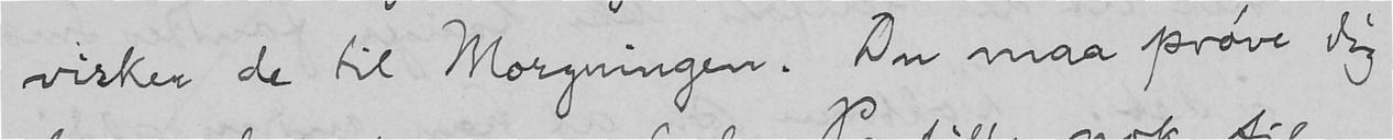virker de til Morgningen. Du maa prøve dig
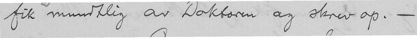fik mundtlig av Doktoren og skrev op. -
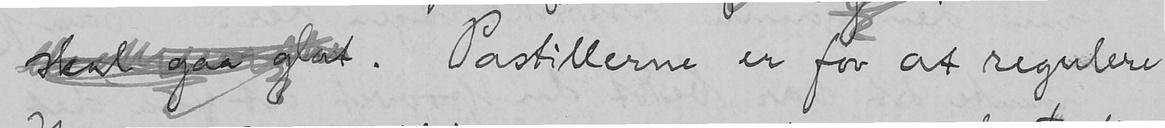skal gaa glat. Pastillerne er for at regulere
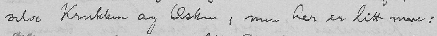selve Krukken og Æsken, men her er litt mere:
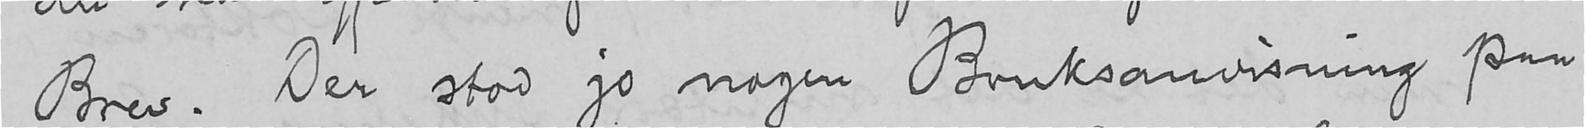Brev. Der stod jo nogen Bruksanvisning paa
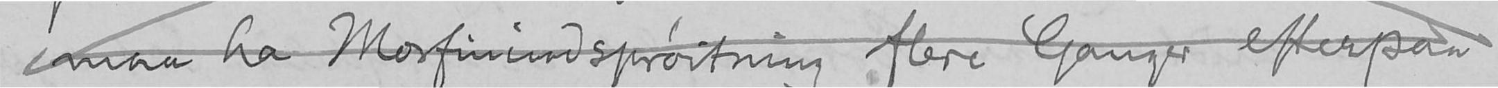maa ha Morfinindsprøitning flere Ganger efterpaa
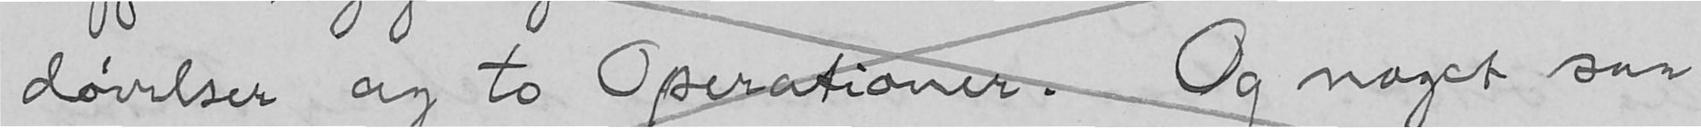døvelser og to Operationer. Og noget saa
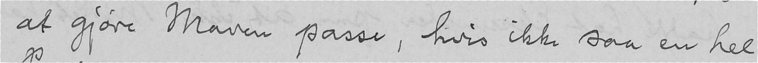at gjøre Maven passe, hvis ikke saa en hel
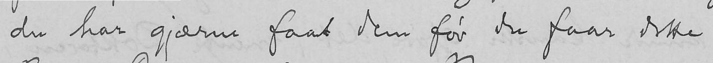du har gjærne faat dem før du faar dette
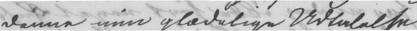denne min glædeligs Udtalelse
